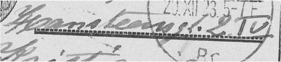Hansteensgt. 2 IV
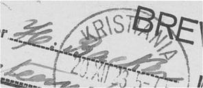H: Backer
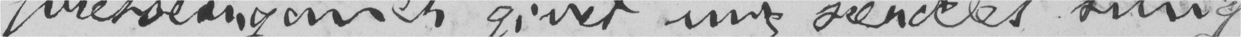presseorganer givet mig særdeles smig-
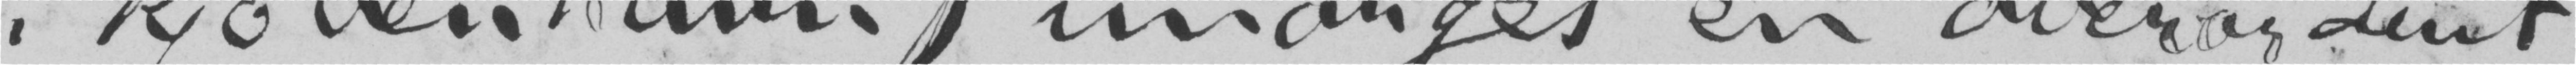i Kjøbenhavn) imorges en overordent-
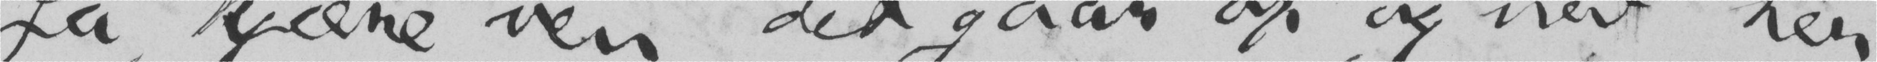Ja, kjære ven, det gaar op og ned her
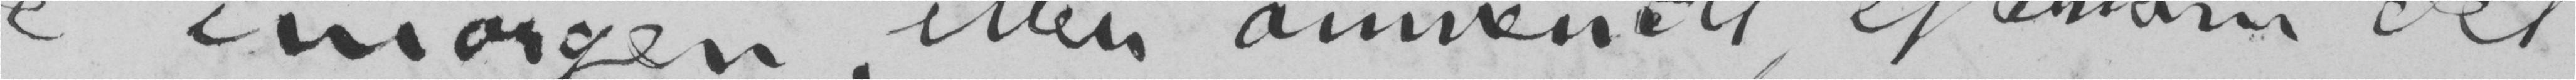imorgen, eller omvendt, eftersom det
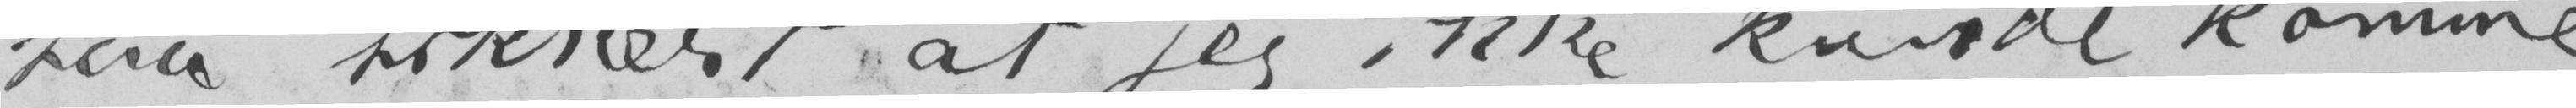saa sikkert at jeg ikke kunde komme
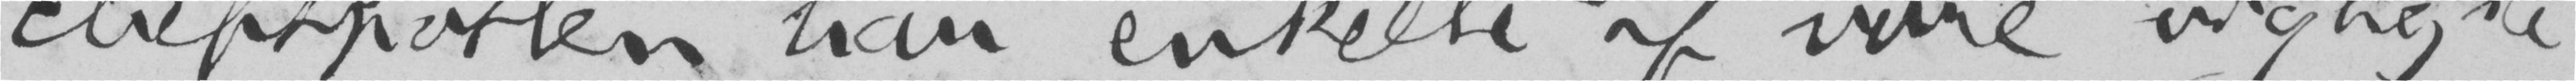chefsposten har enkelte af vore vigtigste
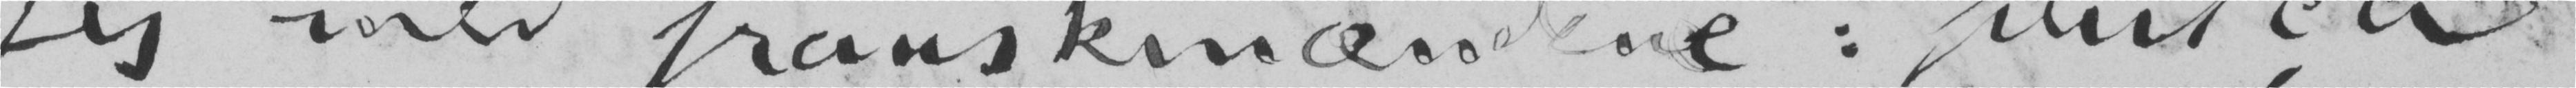jeg med franskmændene: plus ça
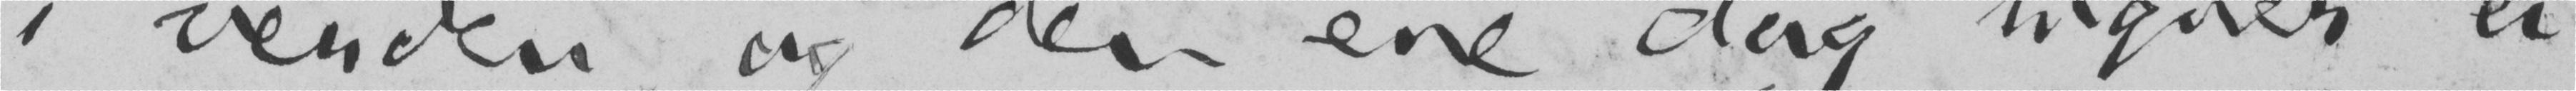i verden, og den ene dag ligner ei
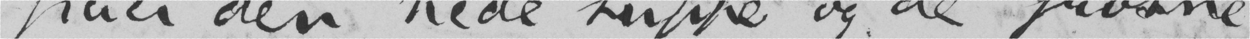paa den hede suppe og de frosne
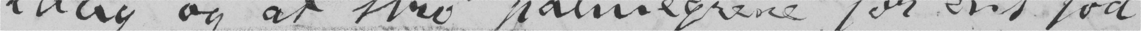idag og at strø palmegrene for ends fod
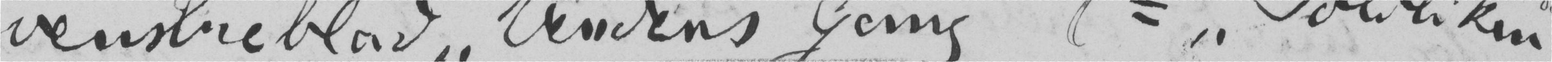venstreblad «Verdens Gang» (= «Politiken»
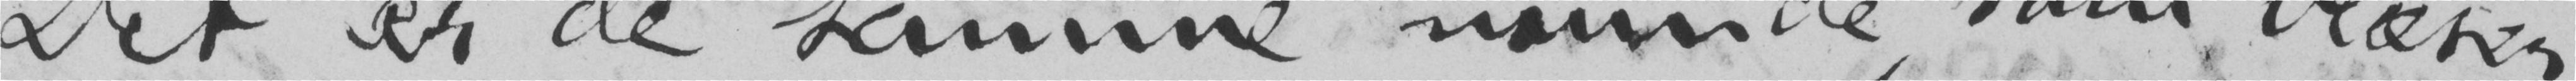Det er de samme munde, som blæser
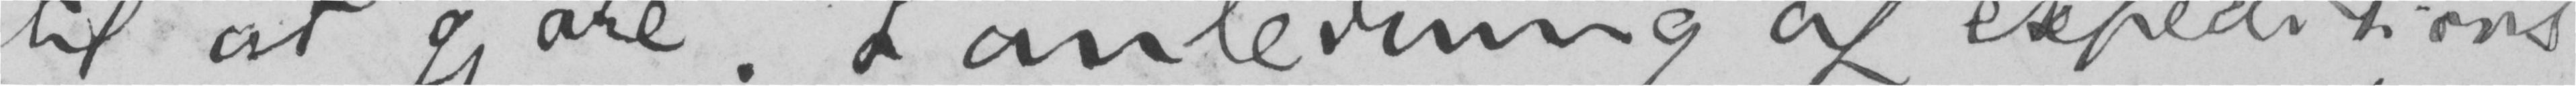til at gjøre. I anledning af expeditions-
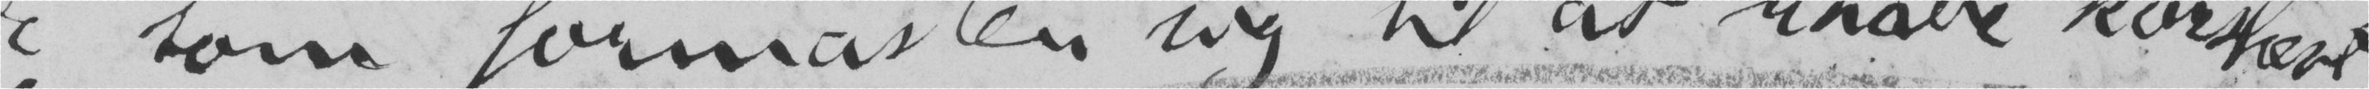som formaster sig til at raabe korsfæst
